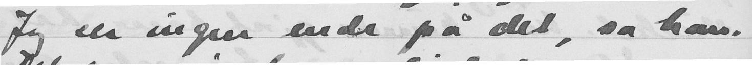Jeg ser ingen ende på det, sa han.
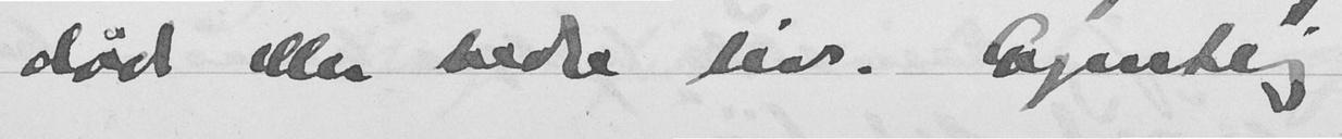død eller bedre liv. - Egentlig
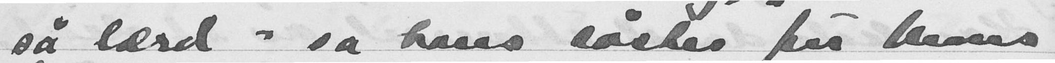så lærd" sa hans søstes fru Mans
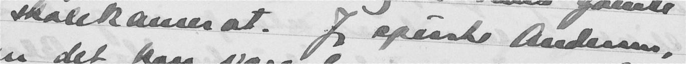skolekamerat. Jeg spurte Andersen,
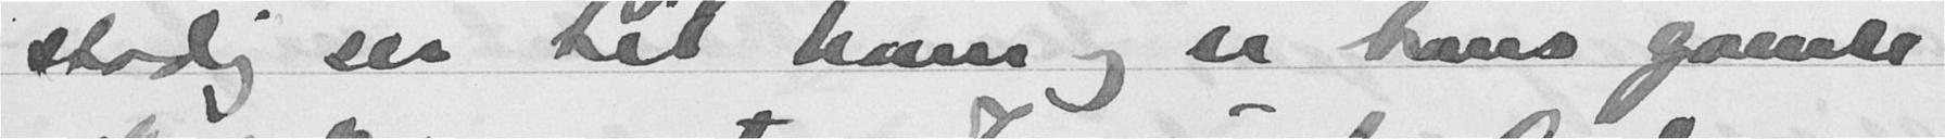stadig ser til ham og er hans gamle
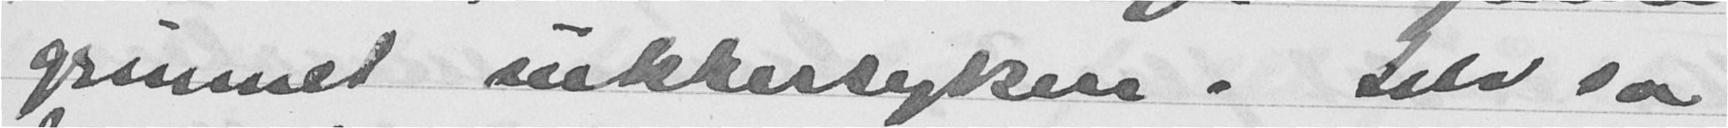grunnet sukkersyken. Selv sa
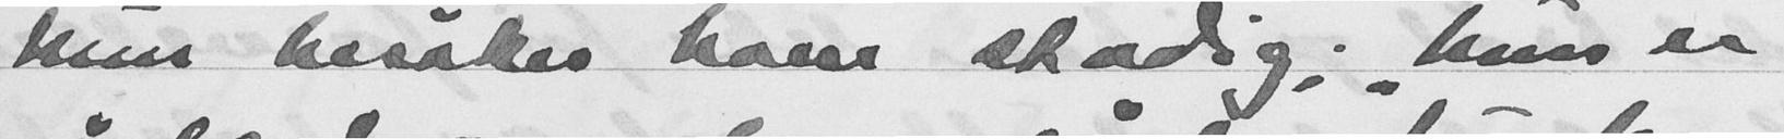hun besøker ham stadig; "hun er
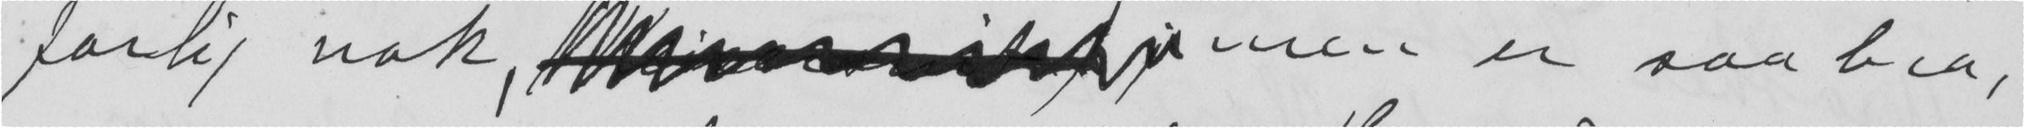farlig nok,  men er saa bra,
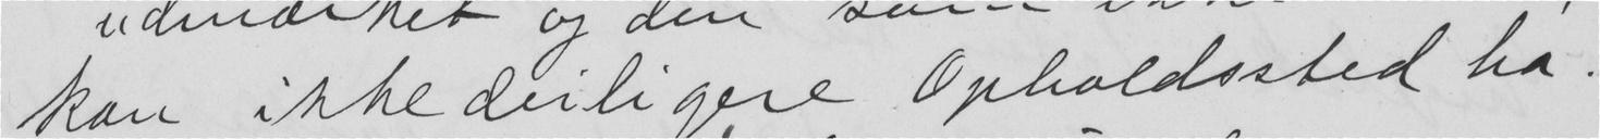kan ikke deiligere Opholdssted ha.
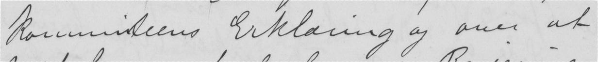kommiteens Erklæring og over at
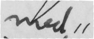med
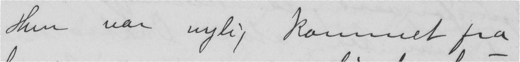Hun var nylig kommet fra
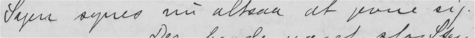Sagen synes nu altsaa at jevne sig.
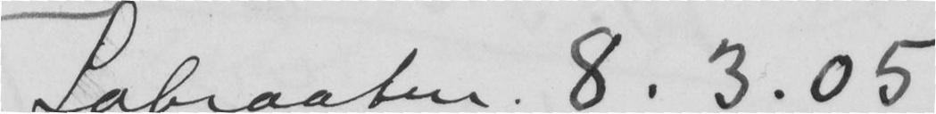Labraaten. 8.3.05
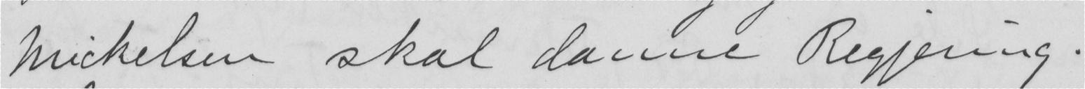Mickelsen skal danne Regjering.
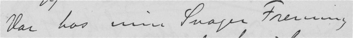Var hos min Svoger Freming
